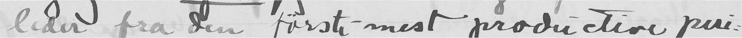leder fra den første - mest productive peri-
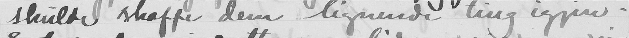skulde skaffe dem lignende ting igjen -
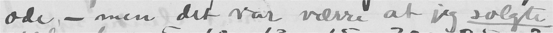ode, - men det var værre at jeg solgte
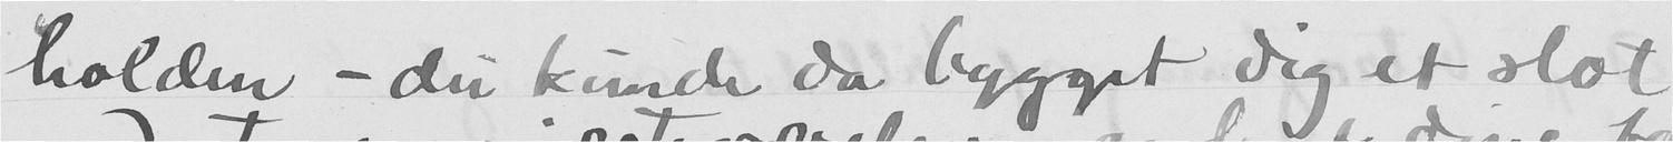holden - du kunde da bygget dig et slot
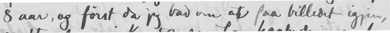8 aar, og først da jeg bad om at faa billedet igjen,
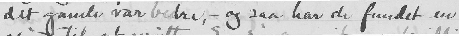det gamle var bedre, - og saa har de fundet en
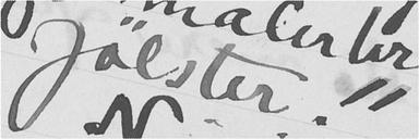Jølster."
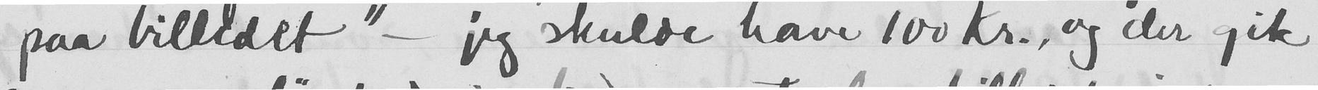paa billidet" - jeg skulde have 100 Kr., og der gik
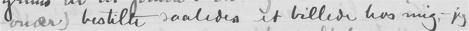onær) bestilte saaledes et billede hos mig, - jeg
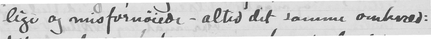lige og misfornøiede - altid det samme omkvæd:
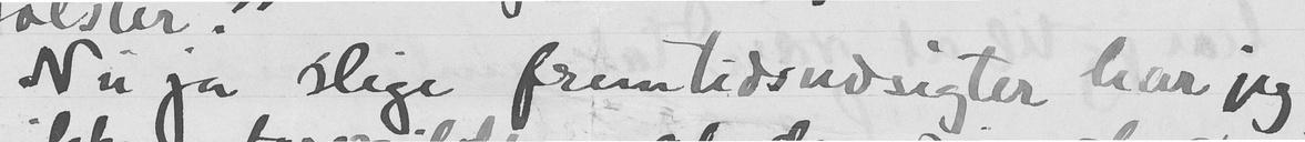Nu ja slige fremtidsudsigter har jeg
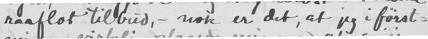raaflot tilbud, - nok er det, at jeg i først-
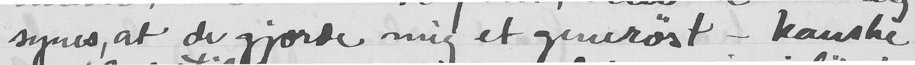synes, at de gjorde mig et generøst - kanske
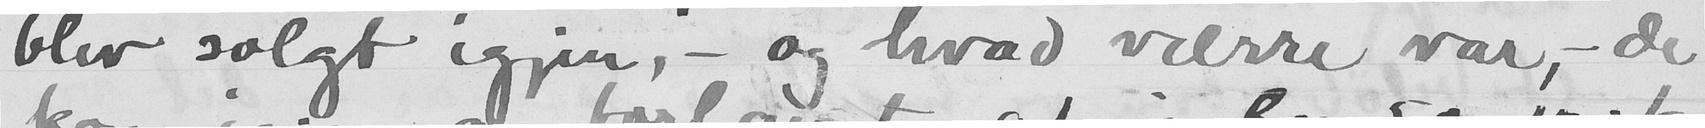blev solgt igjen, - og hvad værre var, - de
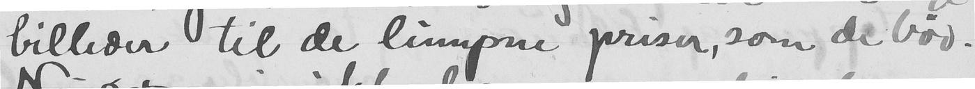billeder til de lumpne priser, som de bød.
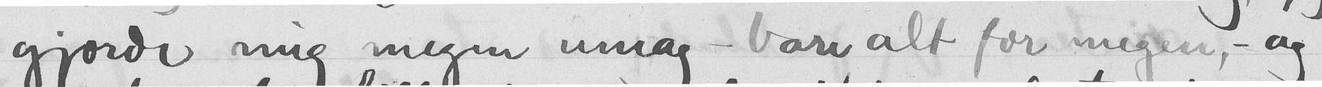gjorde mig megen umag - bare alt for megen, - og
